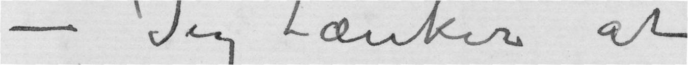– Jeg tænker at
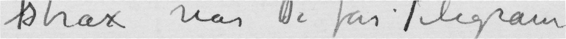strax når De får Telegram
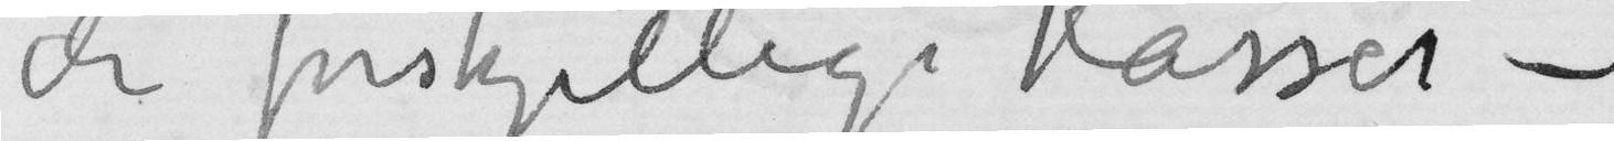de forskjellige Kasser –
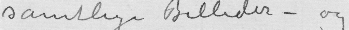samtlige Billeder – og
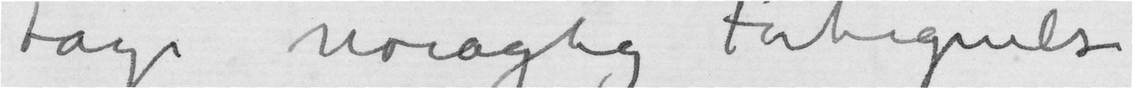tage nøiagtig Fortegnelse
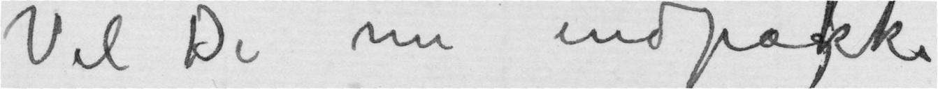Vil De nu indpakke
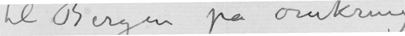til Bergen på omkring
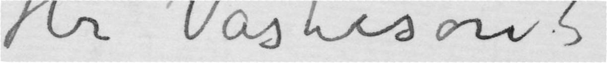Hr Vasteson!
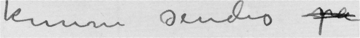kunne sendes på
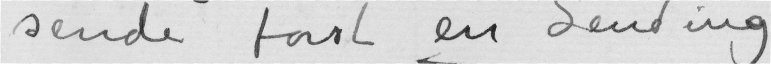sende først en Sending
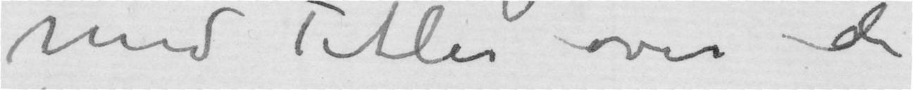med Titler over de
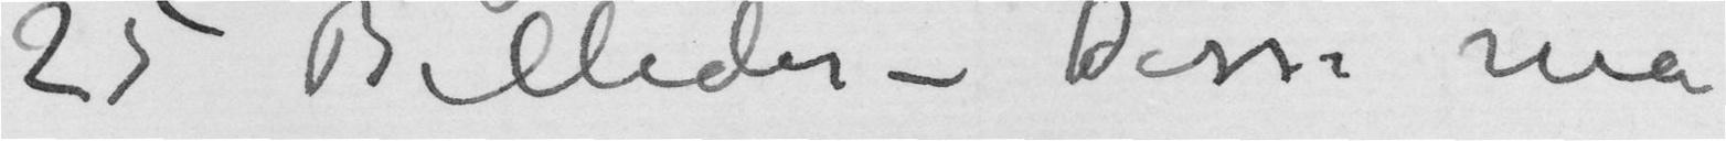25 Billeder – Disse må
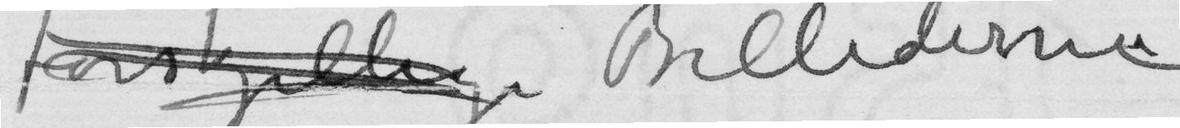forskjellige Billederne
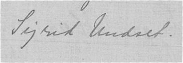Sigrid Undset.
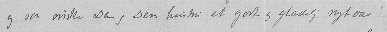og saa ønske Dem og Deres hustru et godt og glædelig nytaar.
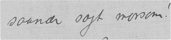saanær sagt morsom!
